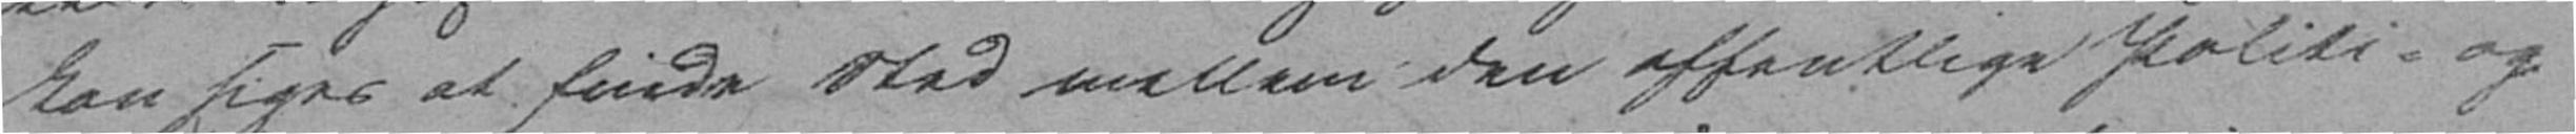kan siges at finde Sted mellem den offentlige Politi- og
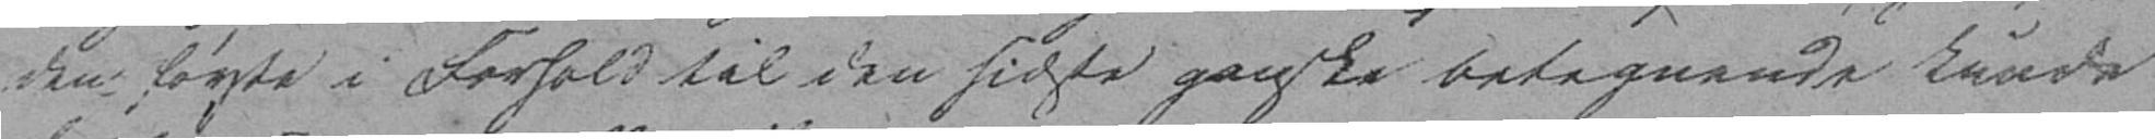den første i Forhold til den sidste ganske betegnende kunde
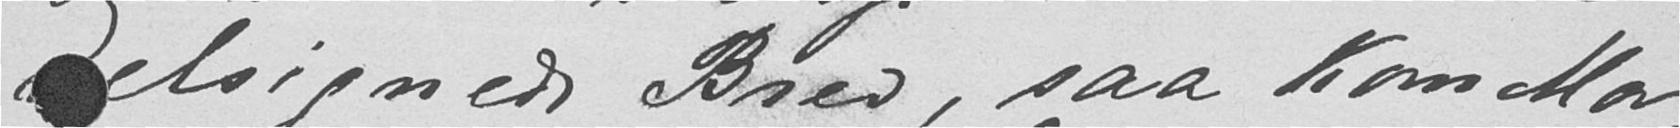velsignede Brev, saa kom Mor-
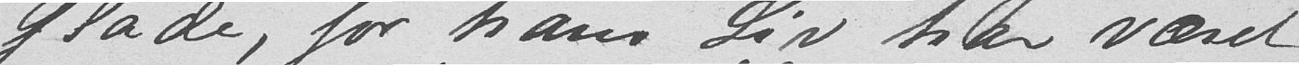glade, for hans Liv har været
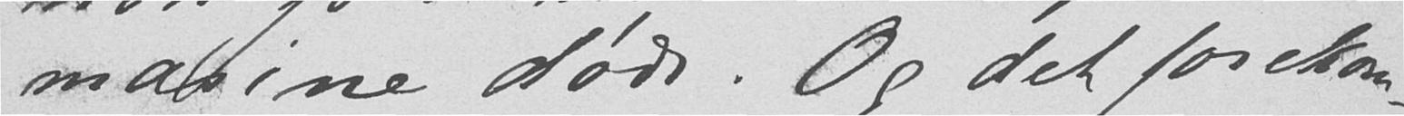masine døde. Og det forekom-
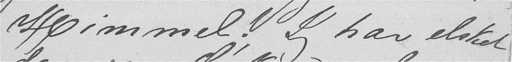Himmel! Jeg har elsket
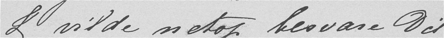Jeg vilde netop besvare Dit
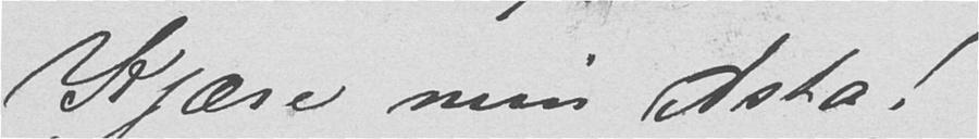Kjære min Asta!
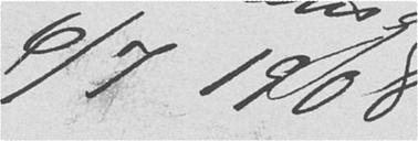6/7 1908
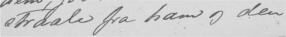straale fra ham og den
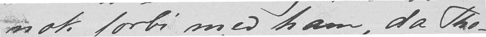nok forbi med ham, da Tho-
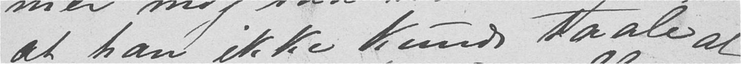at han ikke kunde taale at
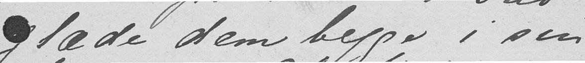glæde dem begge i sin
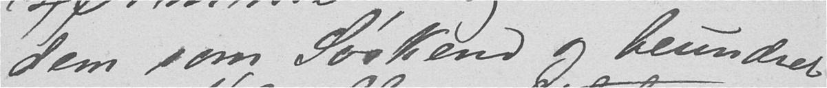dem som Søskend og beundret
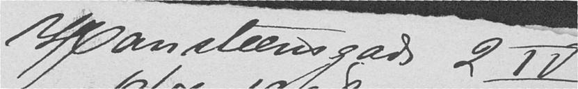Hansteensgade 2 IV
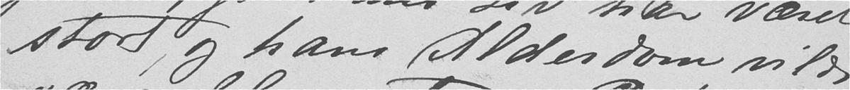stort, og hans Alderdom vilde
